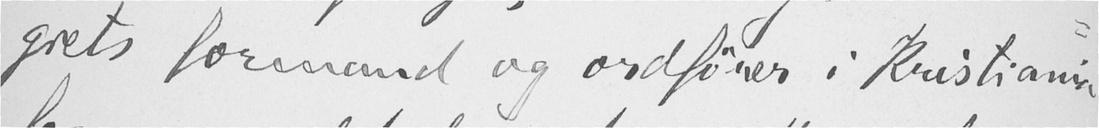giets formand og ordfører i Kristiania
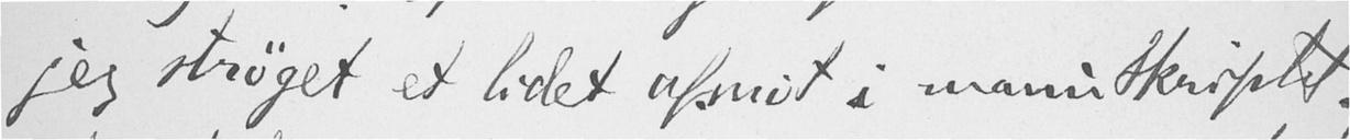jeg strøget et lidet afsnit i manuskriptet.
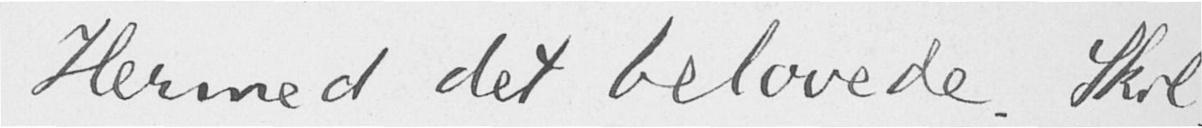Hermed det belovede. Skil-
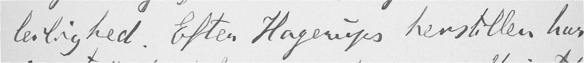leilighed. Efter Hagerups henstillen har
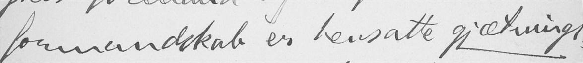formandskab er hensatte gjætnings-
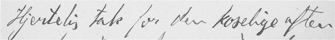Hjertelig tak for den koselige aften
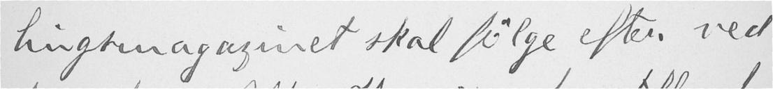lingsmagazinet skal følge efter ved
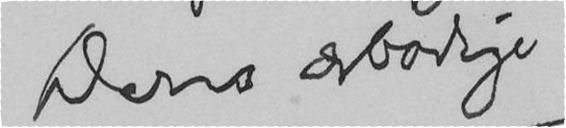Deres ærbødige
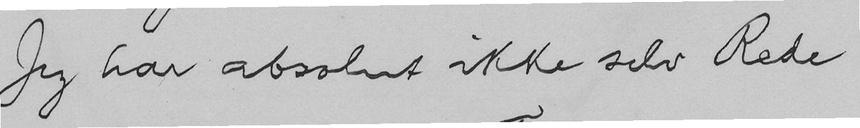Jeg har absolut ikke selv Rede
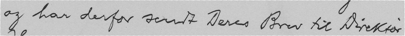og har derfor sendt Deres Brev til Direktør
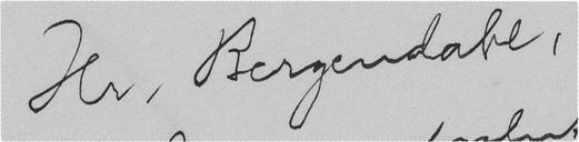Hr. Bergendahl,
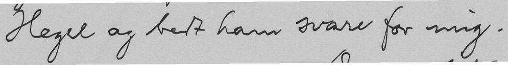Hegel og bedt ham svare for mig.
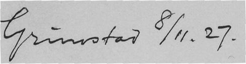Grimstad 8/11. 27.
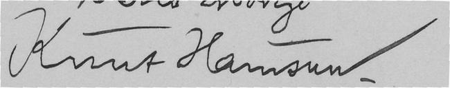Knut Hamsun.
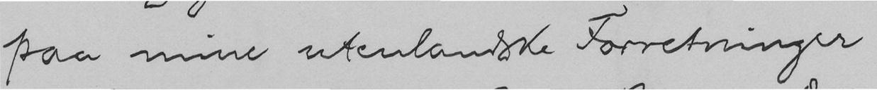paa mine utenlandske Forretninger
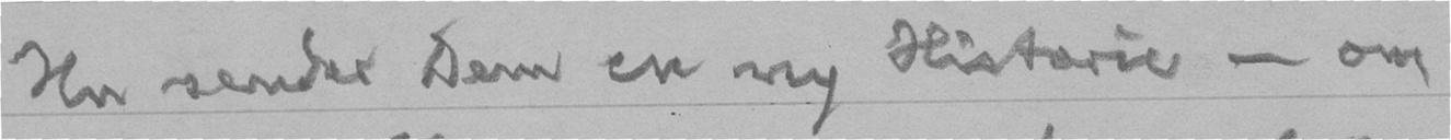Nu sender Dem en ny Historie - om
